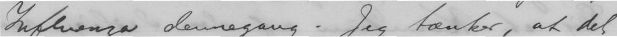Influenza dennegang. Jeg tænker, at det
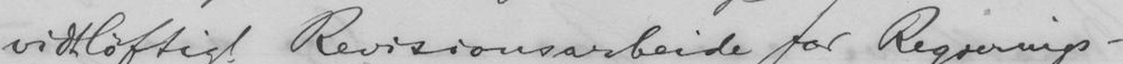vidløftigt Revisionsarbeide for Regjerings-
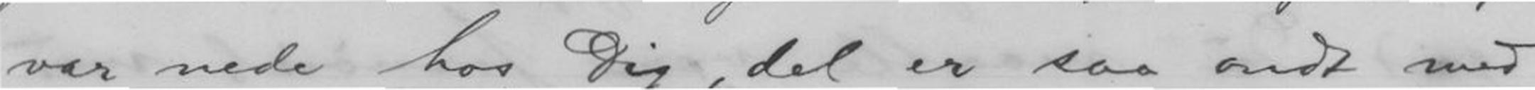var nede hos Dig, det er saa ondt med
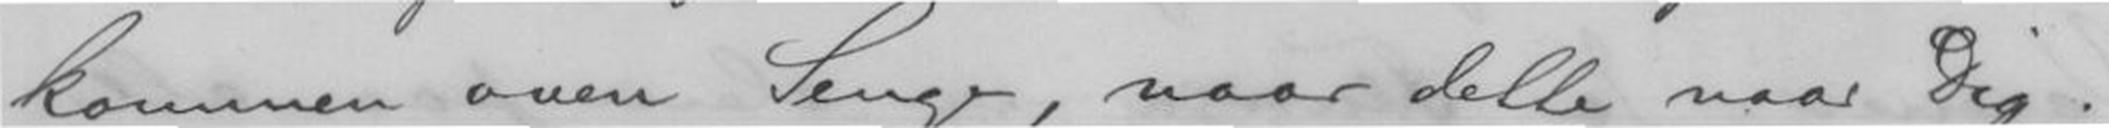kommen oven Senge, naar dette naar Dig.
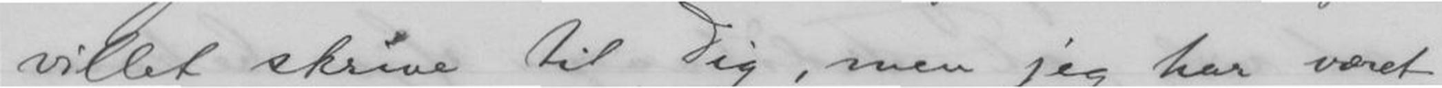villet skrive til Dig, men jeg har været
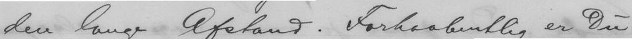den lange Afstand. Forhaabentlig er Du
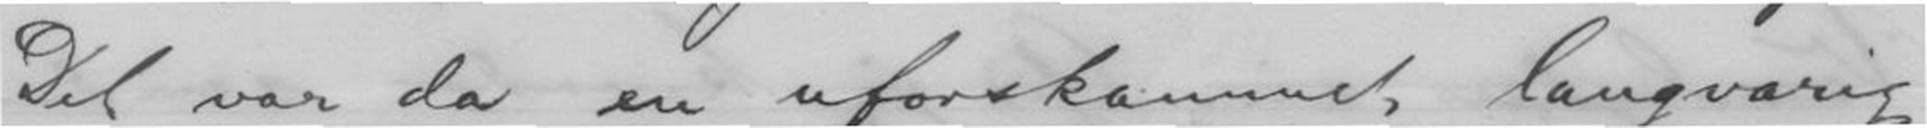Det var da en uforskammet, langvarig
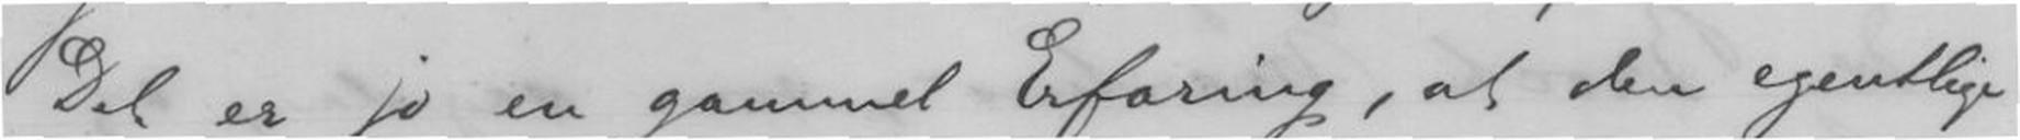Det er jo en gammel Erfaring, at den egentlige
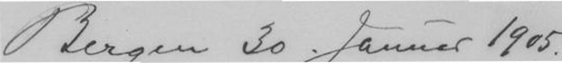Bergen 30. Januar 1905
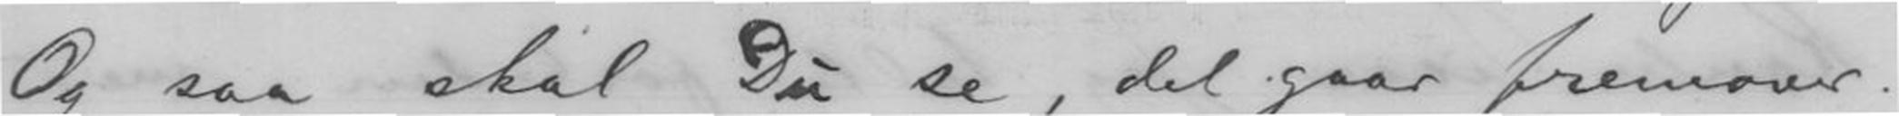Og saa skal Du se, det gaar fremover.
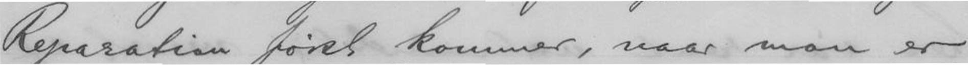Reparation først kommer, naar man er
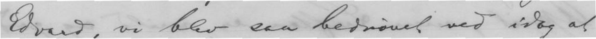Edvard, vi blev saa bedrøvet ved idag at
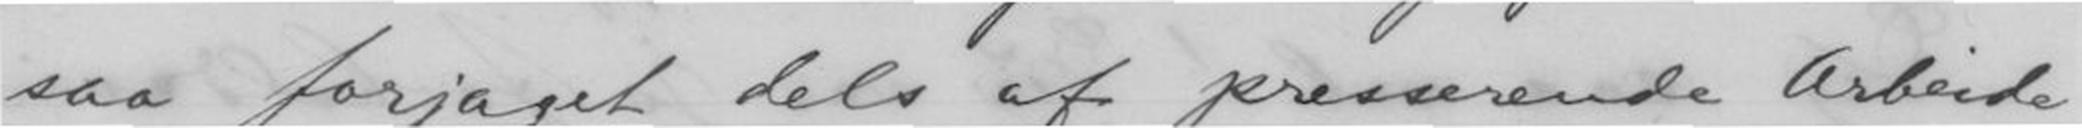saa forjaget dels af presserende Arbeide
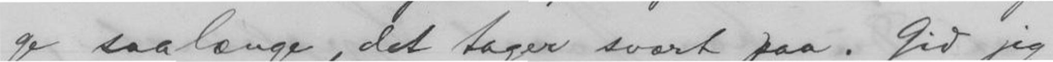ge saalenge, det tager svært paa. Gid jeg
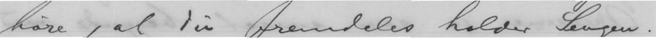høre, at Du fremdeles holder Sengen.
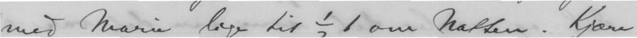med Marie lige til ½1 om Natten. Kjære
